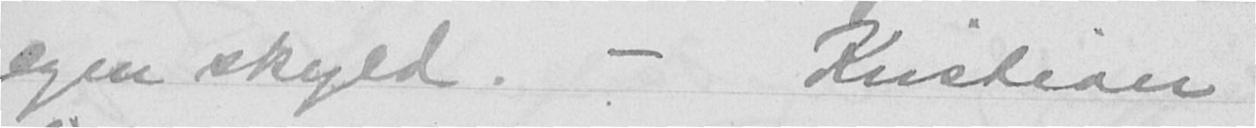egen skyld. - Kristian
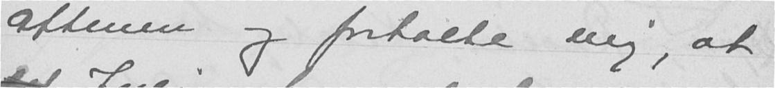aftenen og fortalte mig, at
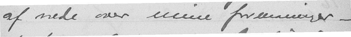af vrede over mine formaninger -
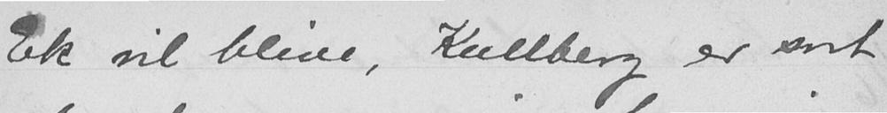Ek vil blive, Kullberg er sort
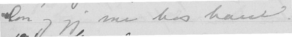Don og jeg var hos ham.
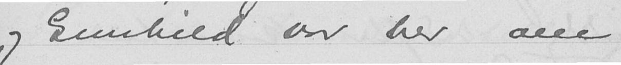og Gunhild var her om
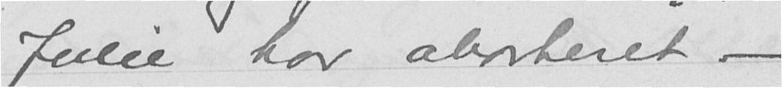Julie har aborteret -
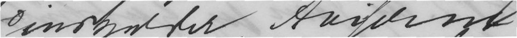indkalder Aviserne
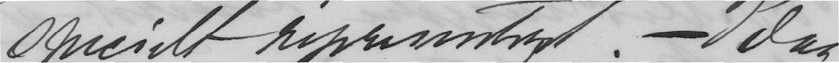spicielt representert. - Idag
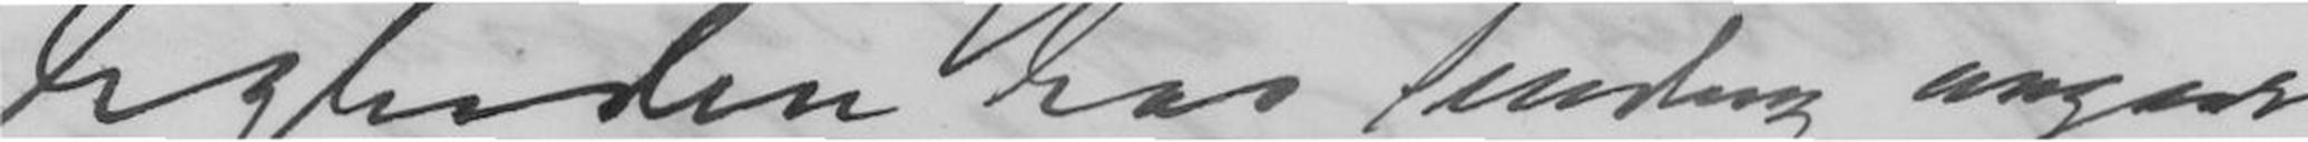ligheden hos Sinding angaar
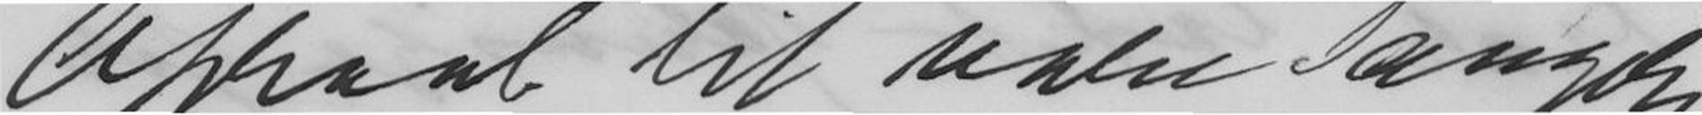Opraab til vore Sangere
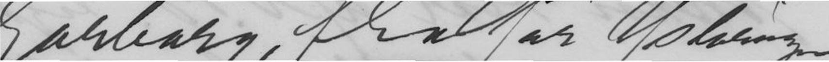Garborg, fEx før Afsløringen,
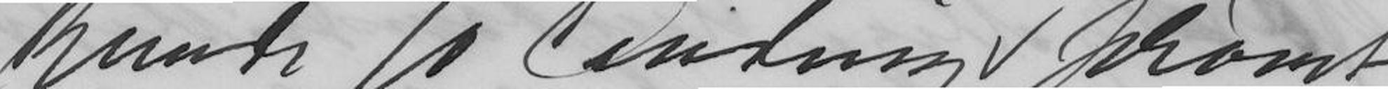kunde jo Sinding  prøvet
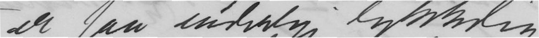er saa inderlig lykkelig
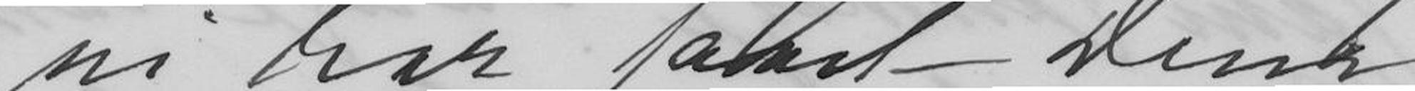vi har faaet Deres
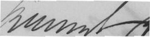kunnet
